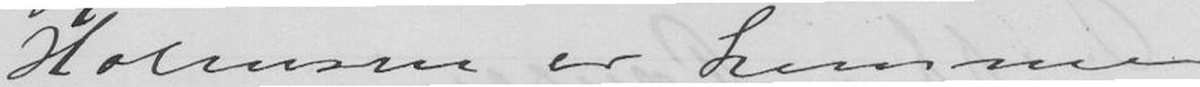Holmsen er kommen.
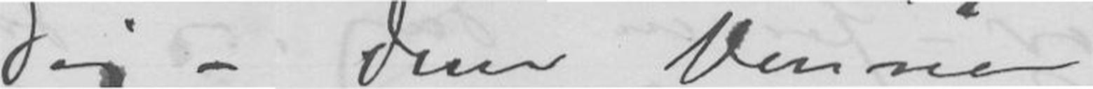Dig - Dine Venner
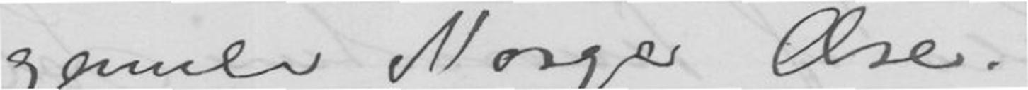gamle Norges Ære.
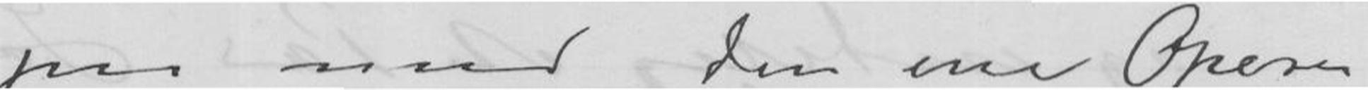paa med den ene Opera
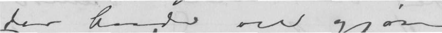der baade vil gjøre
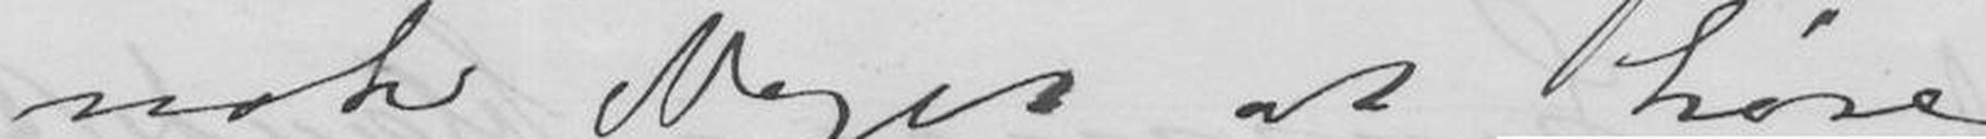nok Meget at høre
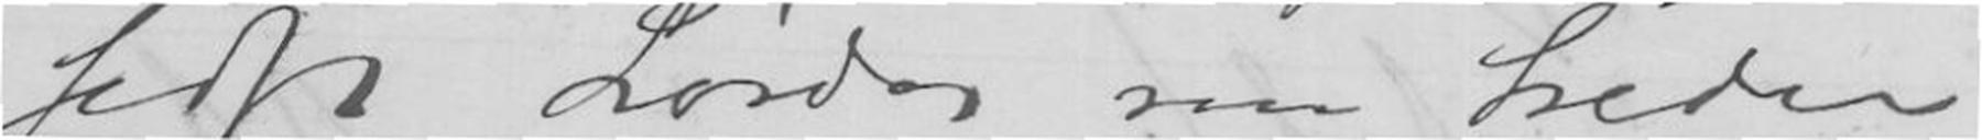sidst Lørdag sin tredie
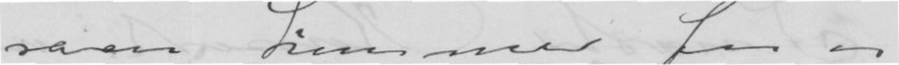raen kommer faa vi
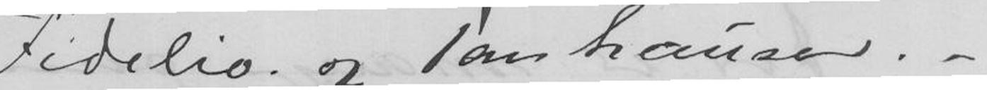Fidelio og Tanhauser. -
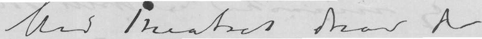Med Theatret drar de
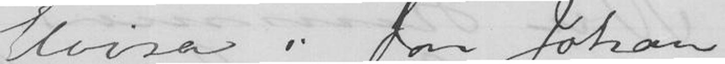Elvira i Don Johan
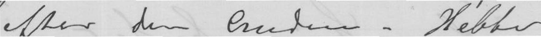efter den Anden. Hebbe
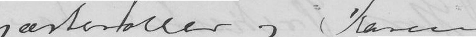Gjæsteroller og Karen
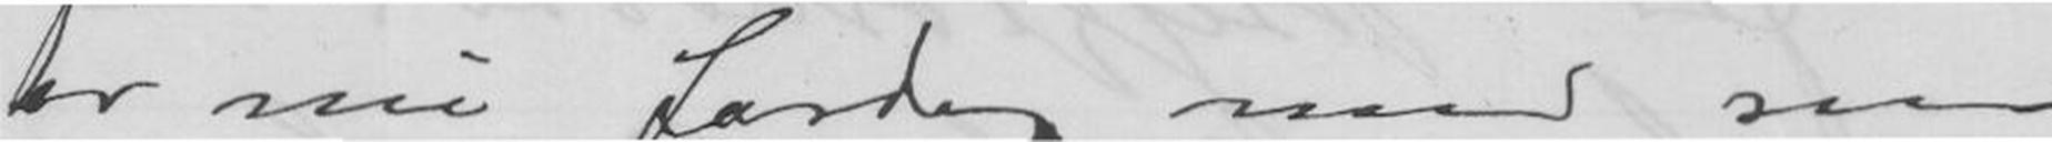er nu færdig med sine
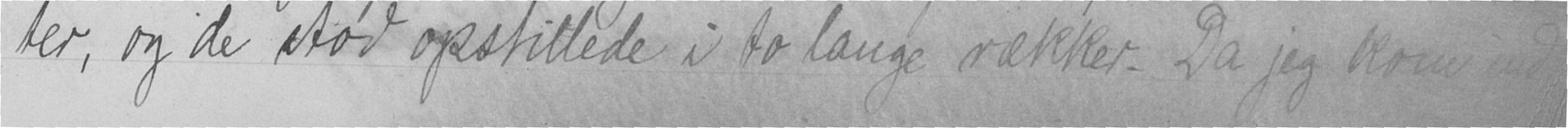ter, og de stod opstillede i to lange rækker. Da jeg kom ind
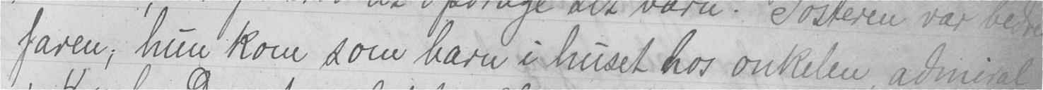faren, hun kom som barn i huset hos onkelen, admiral
